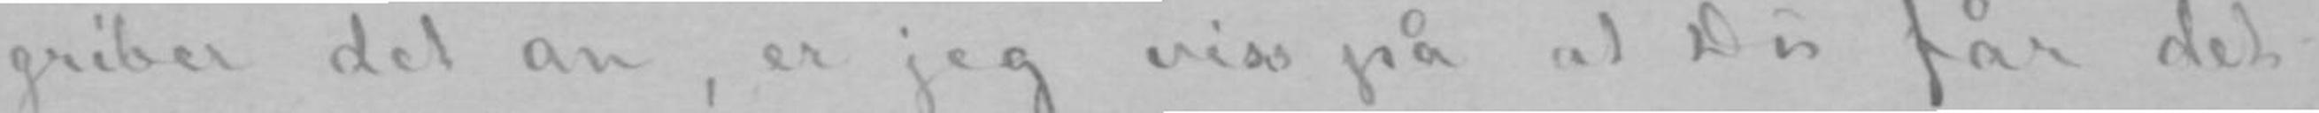griber det an, er jeg viss på at Du får det
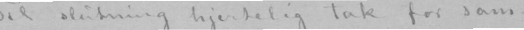Til slutning hjertelig tak for sam-
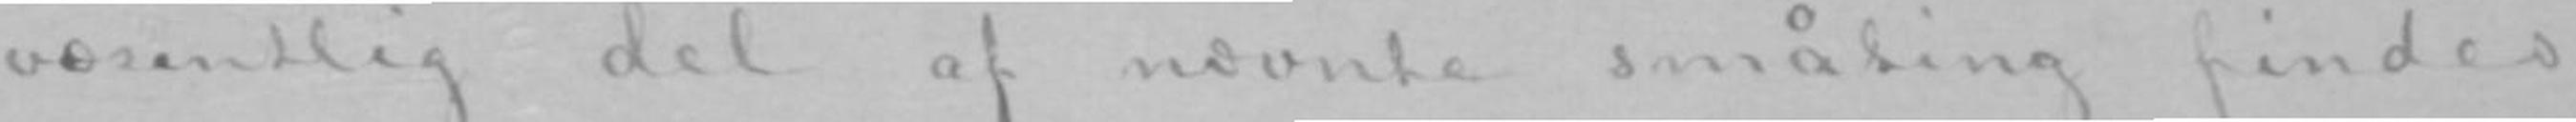væsentlig del af nævnte småting findes
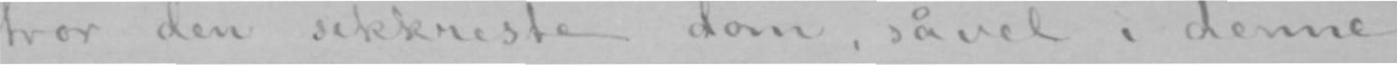tror den sikkreste dom, såvel i denne
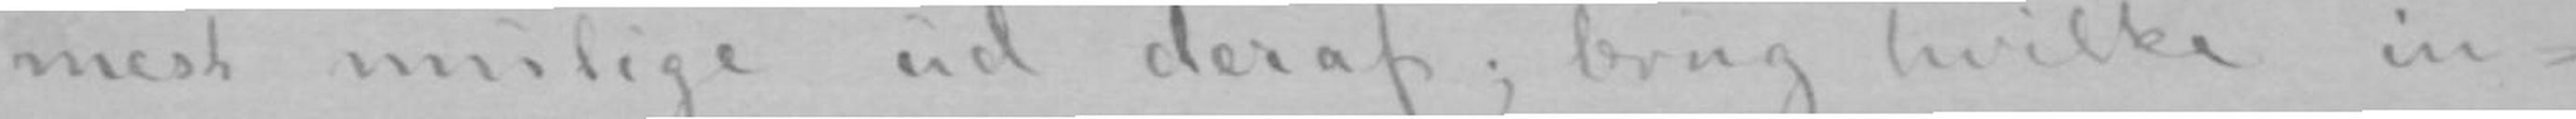mest mulige ud deraf; brug hvilke in-
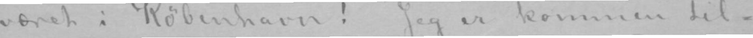været i København! Jeg er kommen til-
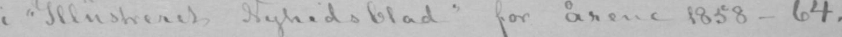i «Illustreret Nyhedsblad» for årene 1858-64.
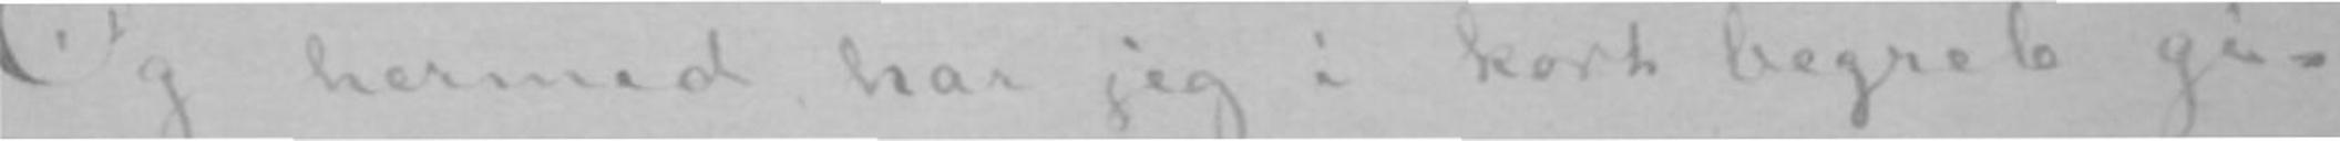Og hermed har jeg i kort begreb gi-
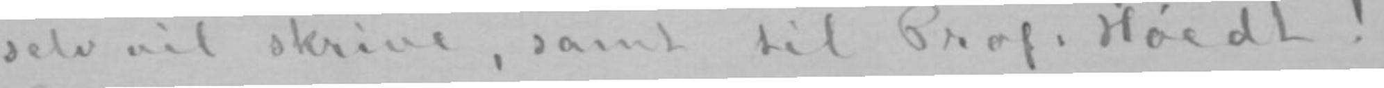selv vil skrive, samt til Prof: Høedt!
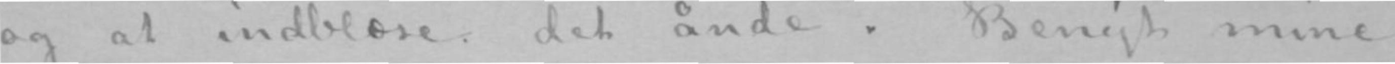og at indblæse det ånde. Benyt mine
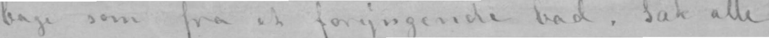bage som fra et foryngende bad. Tak alle
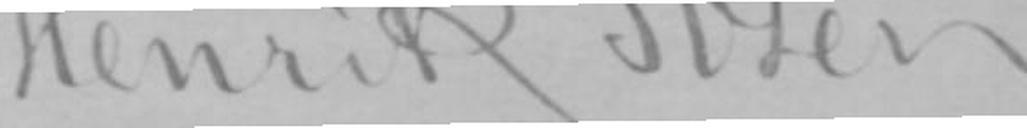Henrik Ibsen.
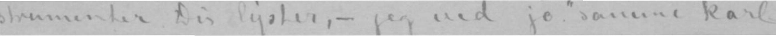strumenter Du lyster,- jeg ved jo «samme karl
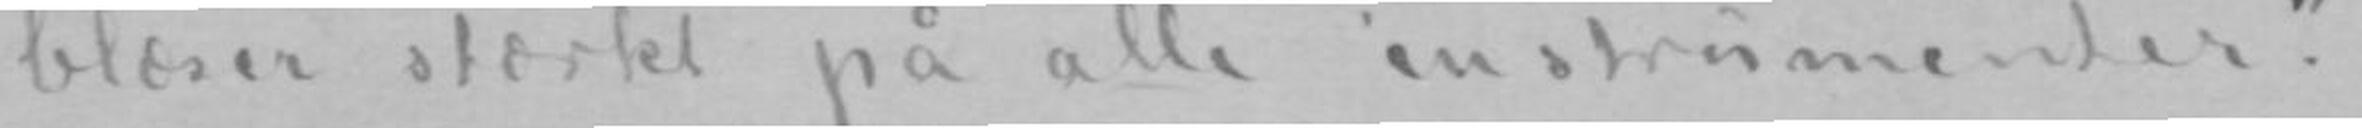blæser stærkt på alle instrumenter».-
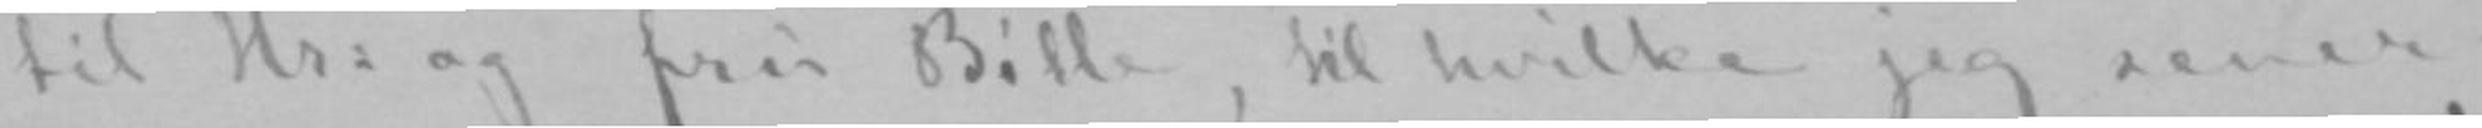til Hr: og fru Bille, til hvilke jeg senere
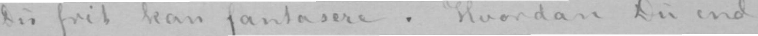Du frit kan fantasere. Hvordan Du end
